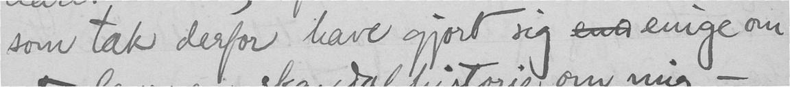som tak derfor have gjort sig  enige om
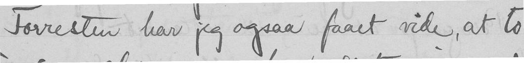Forresten har jeg ogsaa faaet vide, at to
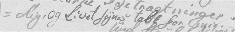-dig, og Livet synes tabt for mig. -
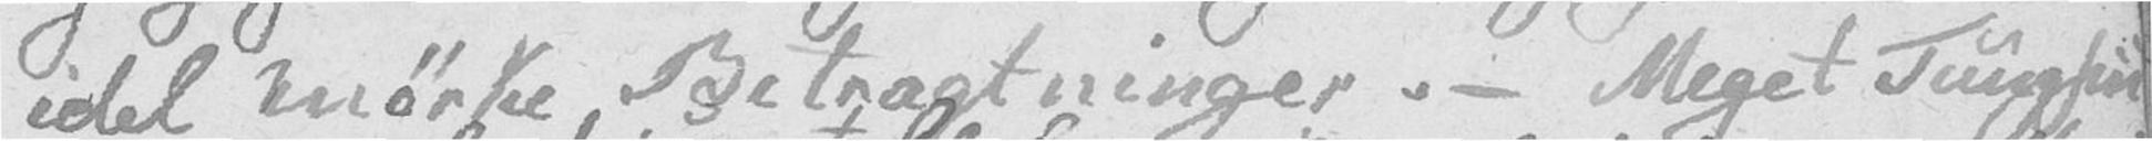idel mørke Betragtninger. – Meget Tungsin
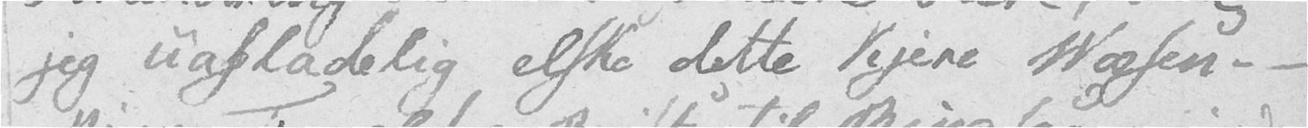jeg uafladelig elske dette kjere Væsen. –
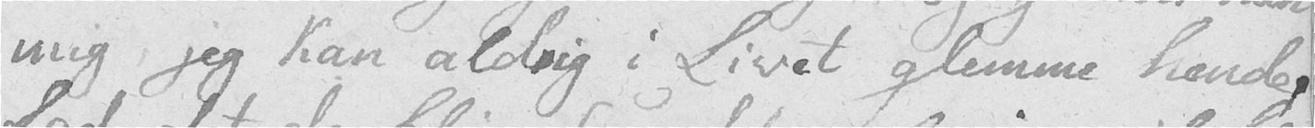mig, jeg kan aldrig i Livet glemme hende,
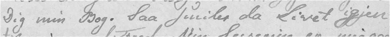Dig min Bog. Saa smiler da Livet igjen
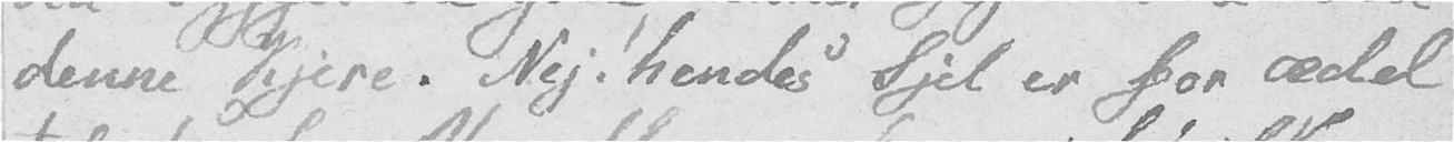denne Kjere. Nej! hendes Sjel er for ædel
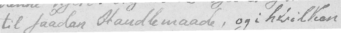til saadan Handlemaade, og i hvilken
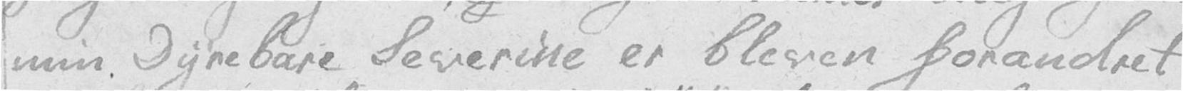min Dyrebare Severine er bleven forandret
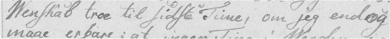Venskab troe til sidste Time, om jeg endog
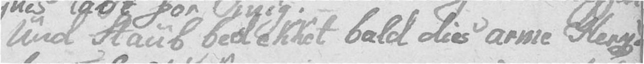Und Staub bedekket bald dies arme Herz
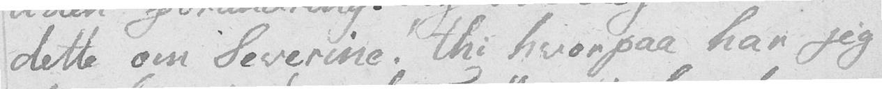dette om Severine! thi hvorpaa har jeg
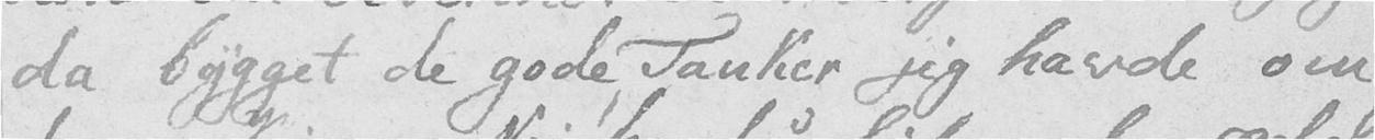da bygget de gode Tanker jeg havde om
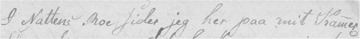I Nattens Roe sider jeg her paa mit Kammer
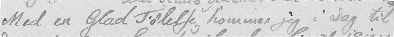Med en Glad Følelse kommer jeg i Dag til
